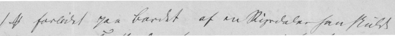1 [Ort] forlidet paa Bordet af en Rigsdaler han skulde
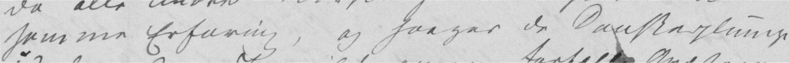samme Erfaring, og saagar de Danske plumpe
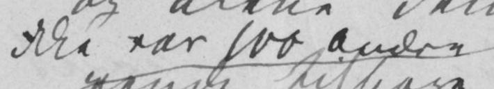ikke var 100 Andre
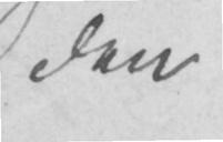den.
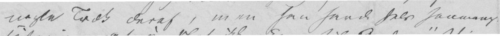nogle Træk deraf, men han havde selv saamange
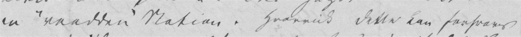en «raadden» Nation. Hvorvidt dette kan forsvares
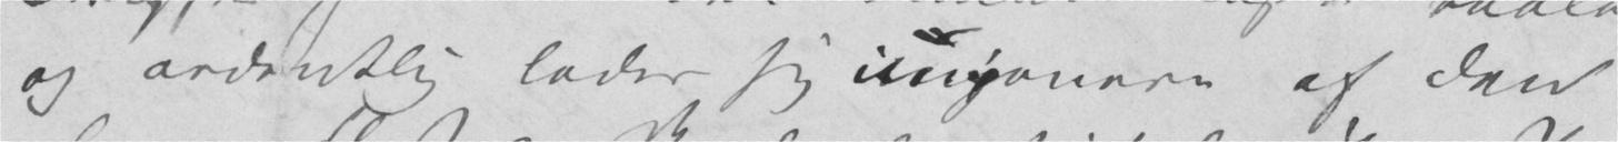og ordentlig lader sig cujonere af den
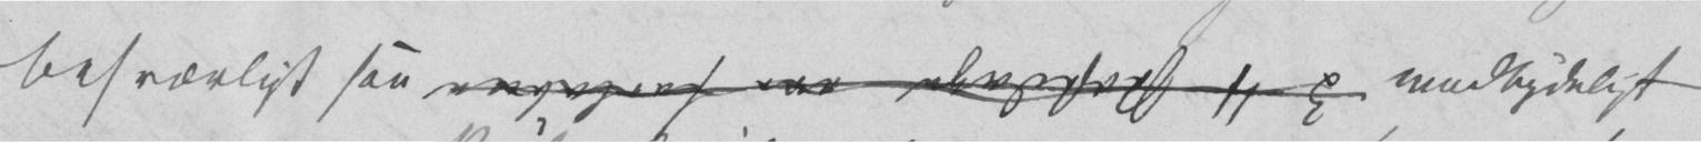besværligt saa modbydeligt
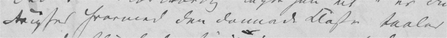Feighed hvormed den dannede Klasse taaler
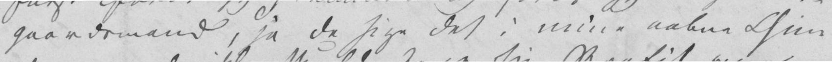gaardsmand, ja de sige det i mine aabne Øine
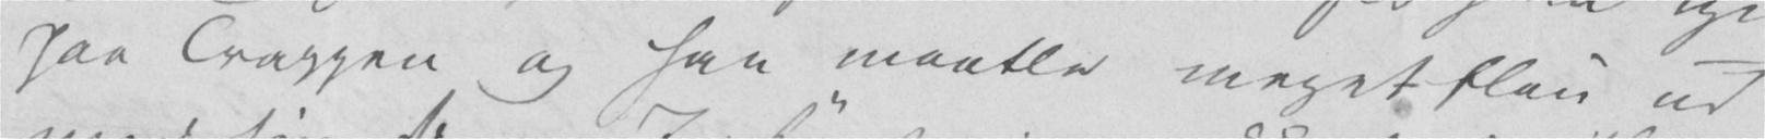paa Trappen og han maatte meget flau ud
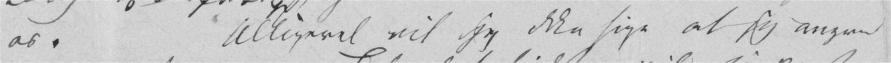os. Alligevel vil jeg ikke sige at jeg angrer
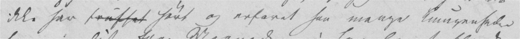ikke har truffet hørt og erfaret saa mange Lumpenheder
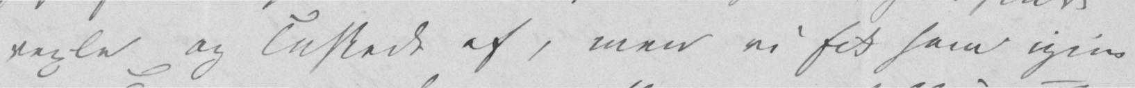vexle og luskede af, men vi fik ham igjen
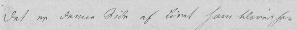Det er denne Side af Livet som bliver saa
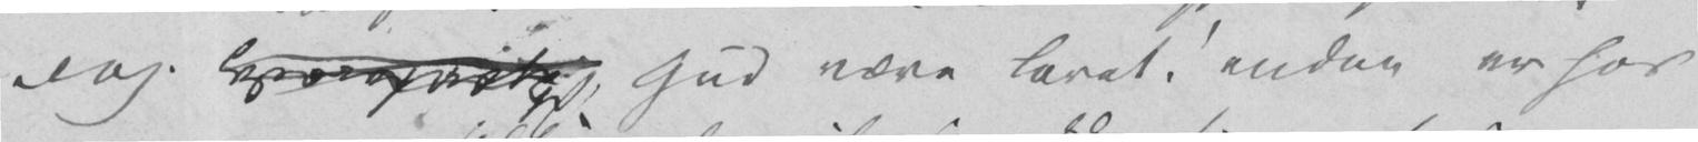dog Gud være lovet! endnu er hos
